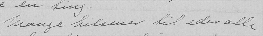Mange hilsener til eder alle
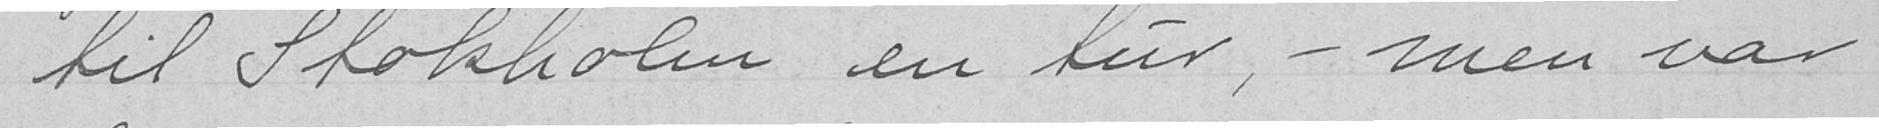til Stokholm en tur, - men var
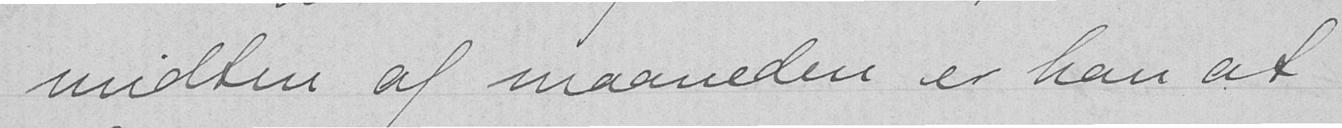midten af maaneden er han at
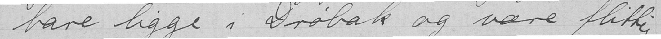bare ligge i Drøbak og være flittig
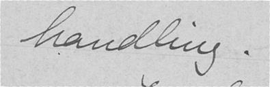handling.
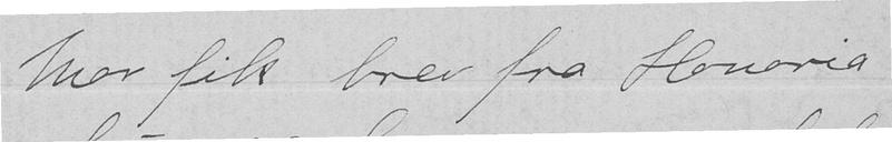Mor fik brev fra Honoria
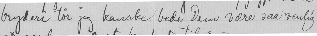bryderi tør jeg kanske bede Dem være saa venlig
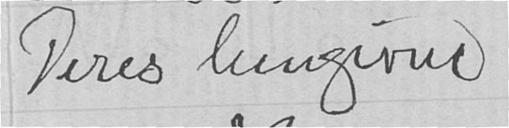Deres hengivne
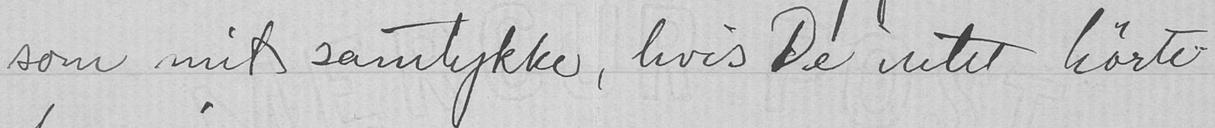som mit samtykke, hvis De intet hørte
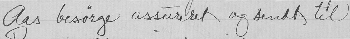Aas besørge assureret og sendt til
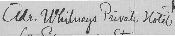Adr. Whilneys Private Hotel
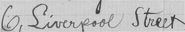6, Liverpool Street
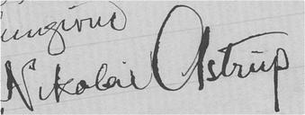Nikolai Astrup
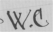W. C
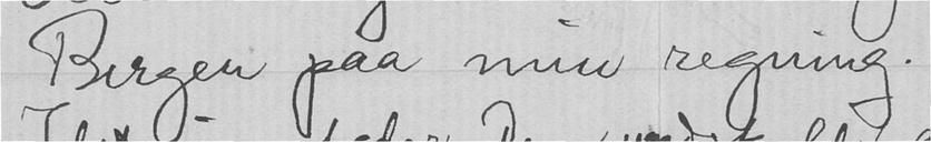Bergen paa min regning.
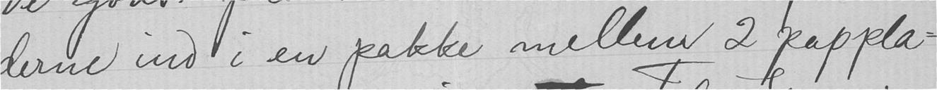derne ind i en pakke mellem 2 pappla-
